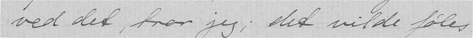ved det, tror jeg; det vilde føles
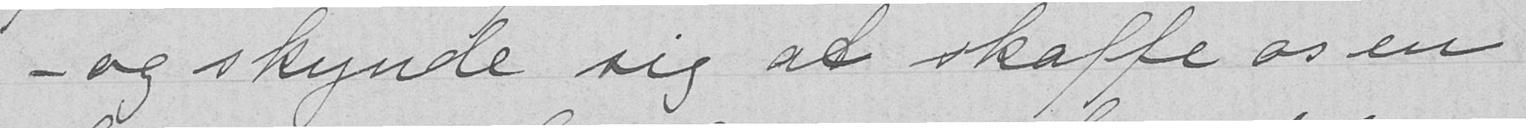- og skynde sig at skaffe os en
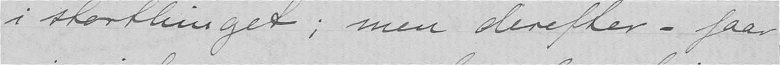i storthinget; men derefter - faar
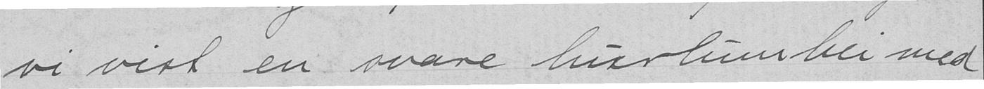vi vist en svare hurlumhei med
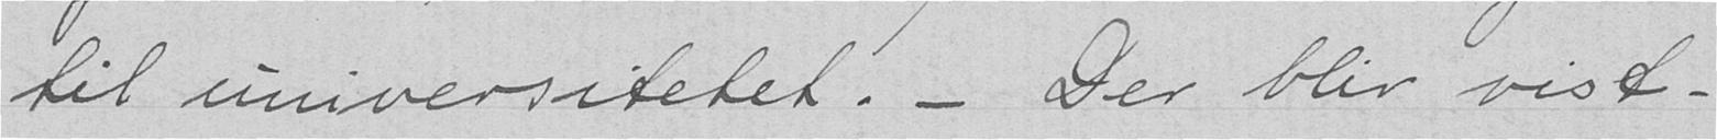til universitetet. - Der blir vist-
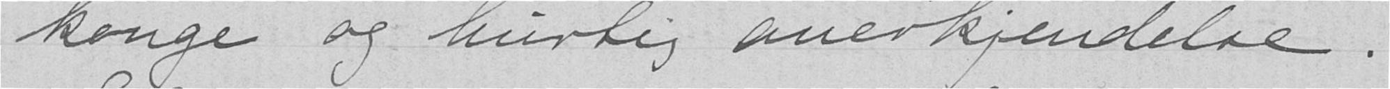konge og hurtig anerkjendelse.
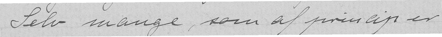Selv mange, som af princip er
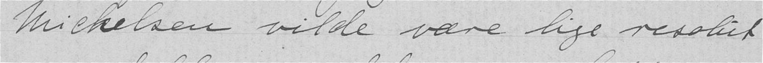Michelsen vilde være lige resolut
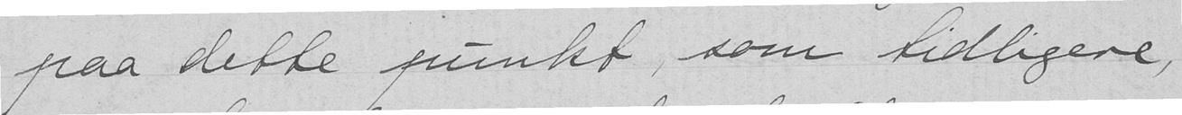paa dette punkt, som tidligere,
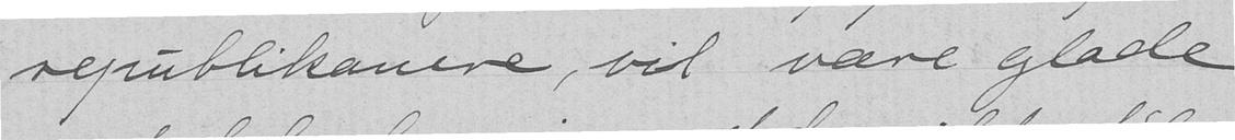republikanere, vil være glade
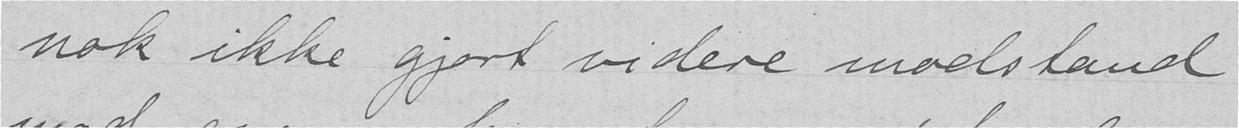nok ikke gjort videre modstand
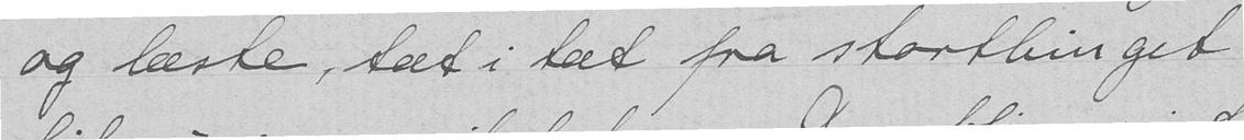og læste, tæt i tæt fra storthinget
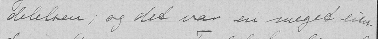delelsen; og det var en meget eien-
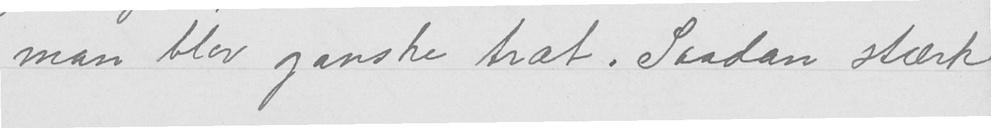man blev ganske træt. Saadan stærk
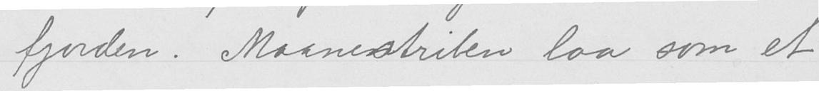fjorden. Maanestriben laa som et
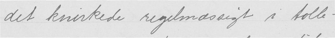det knirkede regelmæssigt i tolle-
